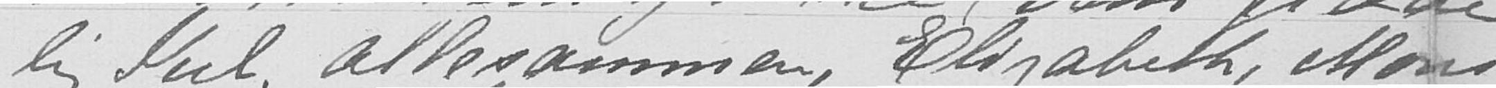lig Jul allesammen, Elizabett, Mons
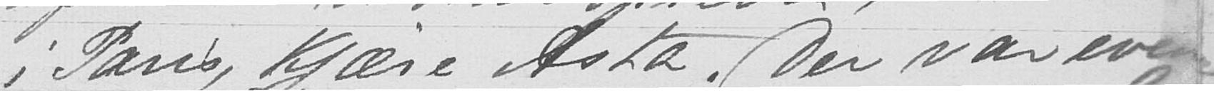i Paris, Kjære Asta. Der var even-
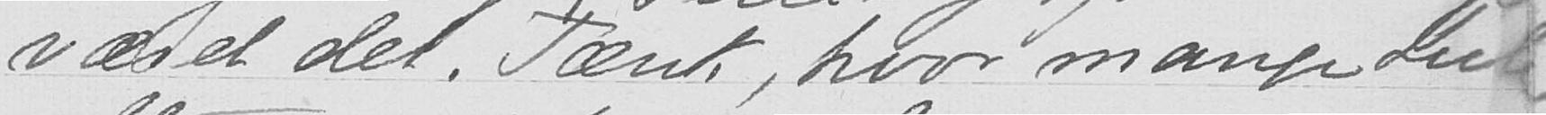været det. Tænk, hvor mange Jule-
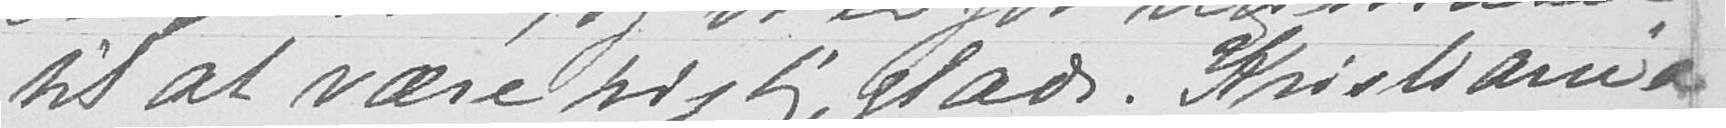til at være rigtig glade. Kristiania
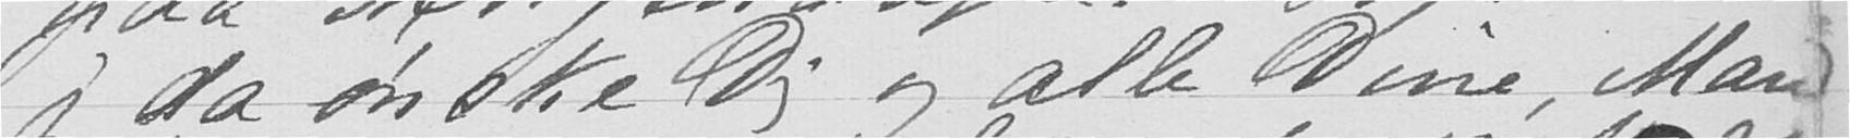jeg da ønske Dig og alle Dine, Mand
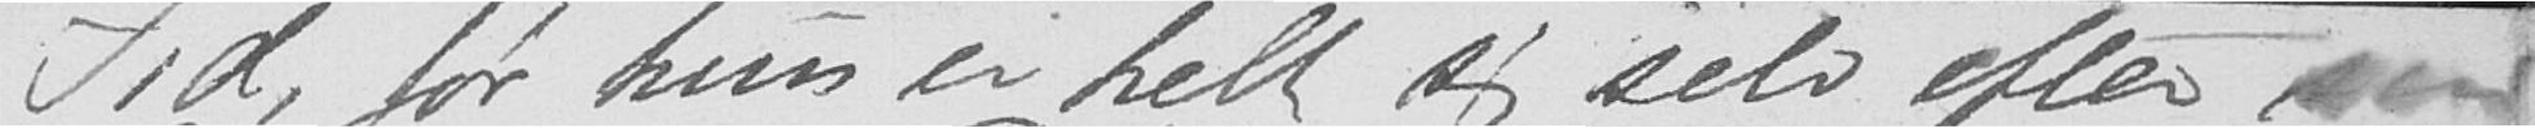Tid, før hun er helt sig selv efter en
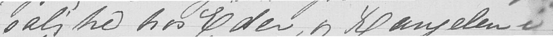salghed hos Eder, og Rangelen i
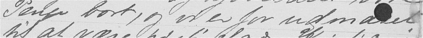Penge bort, og vi er for udmaset
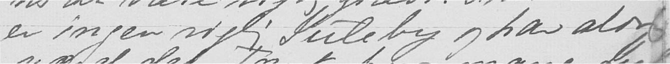er ingen rigtig Juleby og har aldrig
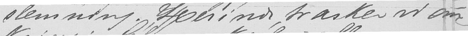stemning. Herinde trasker vi om-
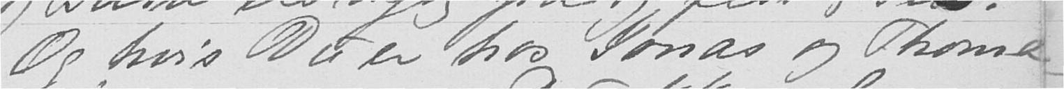Og hvis Du er hos Jonas og Thoma-
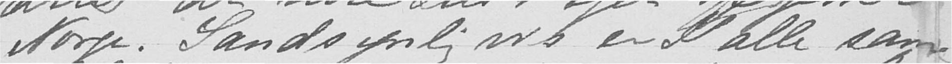Norge. Sandsynligvis er I alle sam-
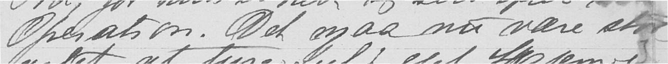Operation. Det maa nu være stor-
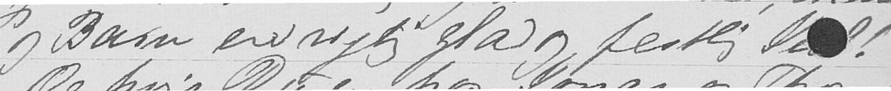og Barn er rigtig glad og festlig Jul!
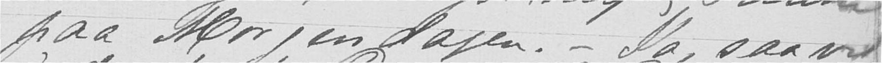paa Morgendagen. Ja, saa vil
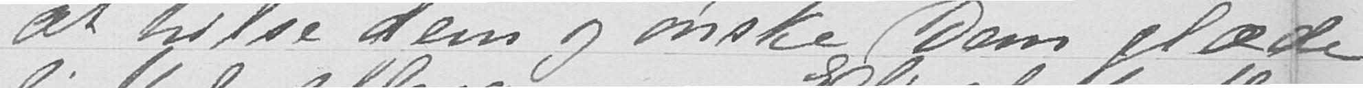at hilse dem og ønske Dem glæde-
